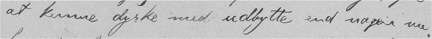at kunne dyrke med udbytte end nogen nu-
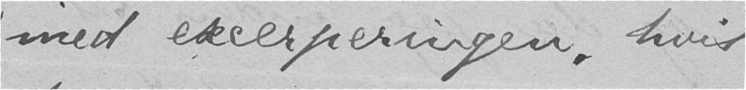med excerperingen, hvis
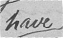have
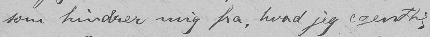som hindrer mig fra, hvad jeg egentlig
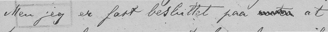Men jeg er fast besluttet paa  at
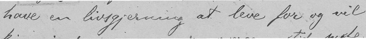have en livsgjerning at leve for og vil
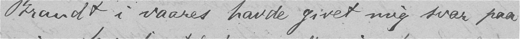Brandt i vaares havde givet mig svar paa
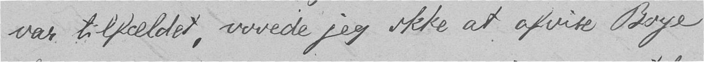var tilfældet, vovede jeg ikke at afvise Boye
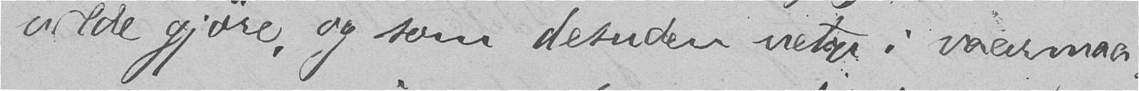vilde gjøre, og som desuden netop i vaarmaa-
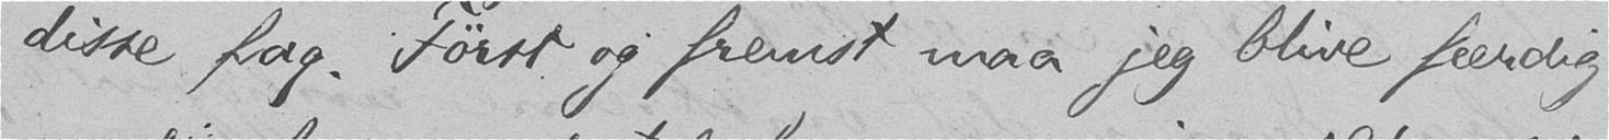disse fag. Først og fremst maa jeg blive færdig
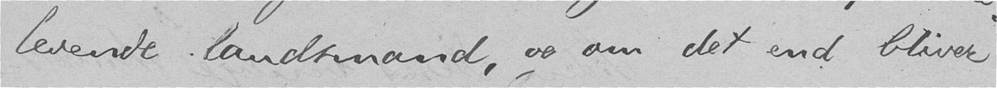levende landsmand, og om det end bliver
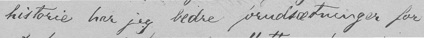historie har jeg bedre forudsætninger for
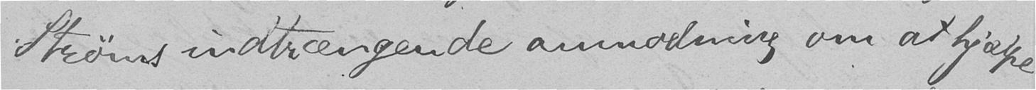Strøms indtrængende anmodning om at hjælpe
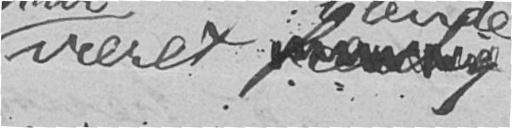været
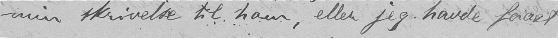min skrivelse til ham, eller jeg havde faaet
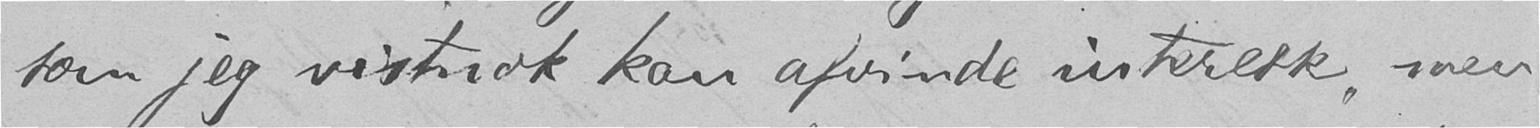som jeg vistnok kan afvinde interesse, men
